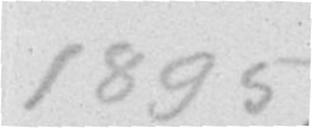1895.
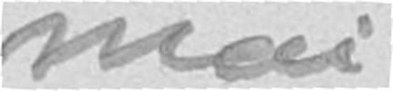mai
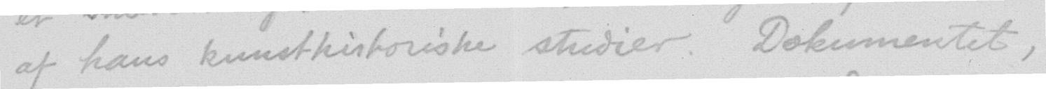af hans kunsthistoriske studier. Dokumentet,
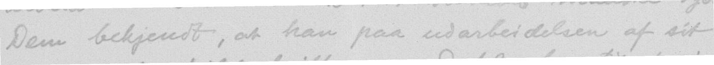Dem bekjendt, at han paa udarbeidelsen af sit
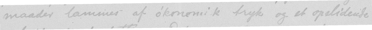maader lammes af økonomisk tryk og et opslidende
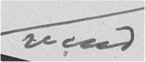vend
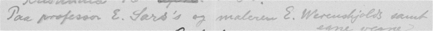Paa professor E. Sars's og maleren E. Werenskjolds samt
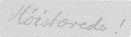Høistærede!
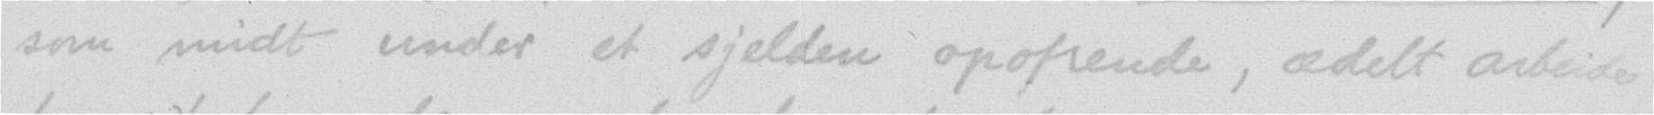som midt under et sjelden opofrende, ædelt arbeide
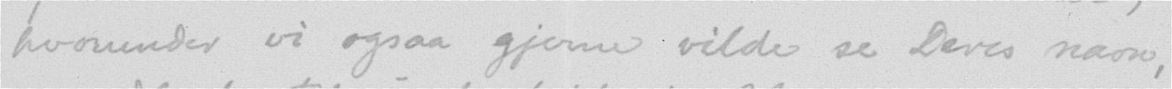hvorunder vi ogsaa gjerne vilde se Deres navn,
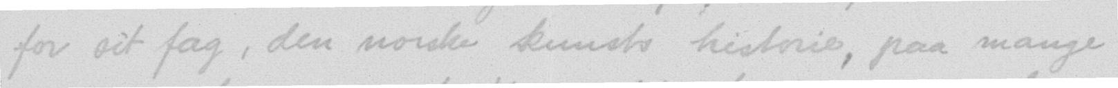for sit fag, den norske kunsts historie, paa mange
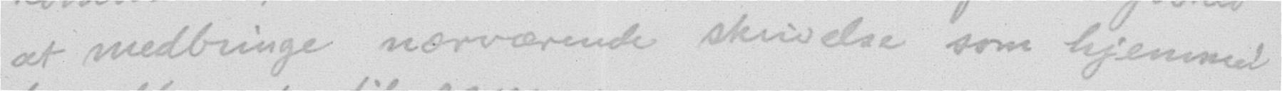at medbringe nærværende skrivelse som hjemmel
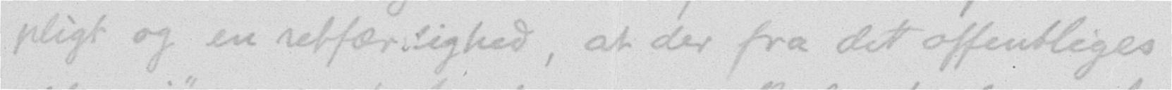pligt og en retfærdighed, at der fra det offentliges
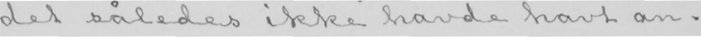det således ikke havde havt an-
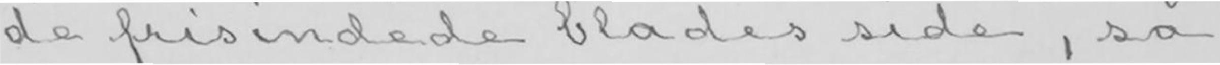de frisindede blades side, så
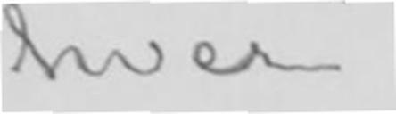hver.
